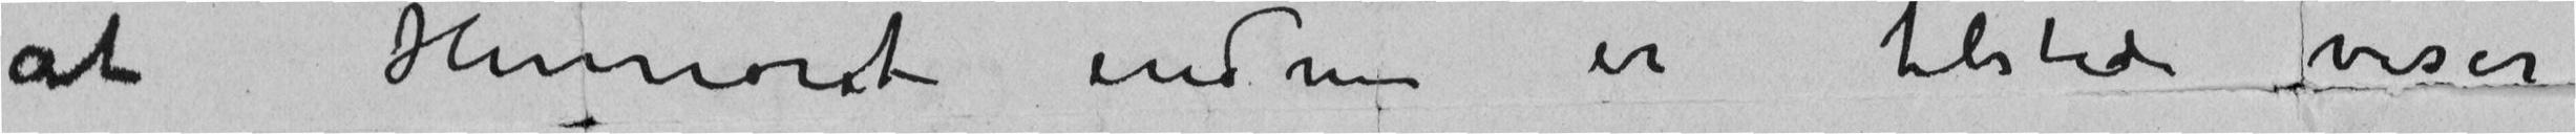at Humøret endnu er tilstede viser
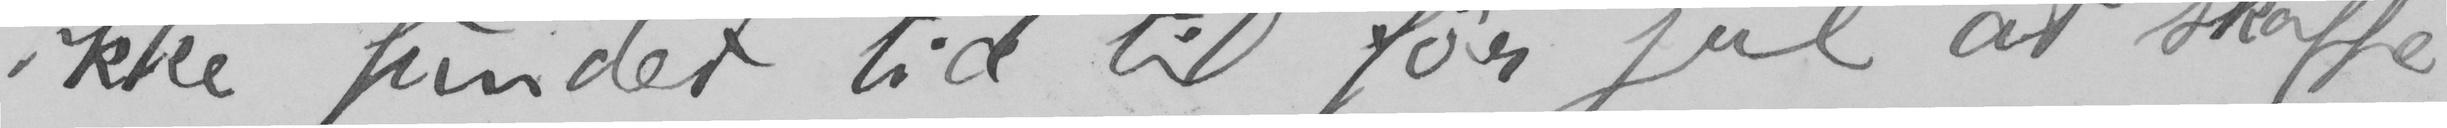ikke fundet tid til før jul at skaffe
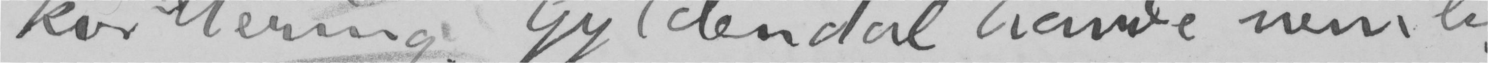kvittering. Gyldendal havde nemlig
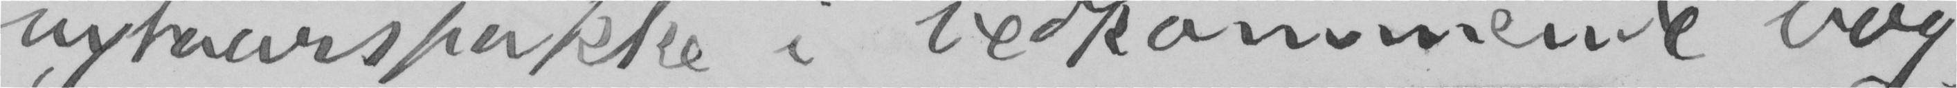nytaarspakke i vedkommende bog-
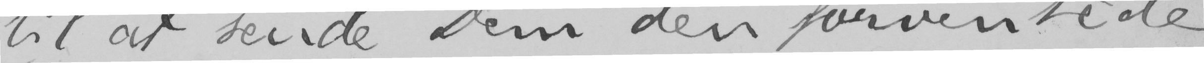til at sende Dem den forventede
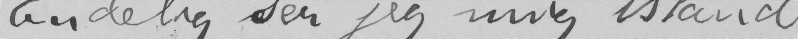Endelig ser jeg mig istand
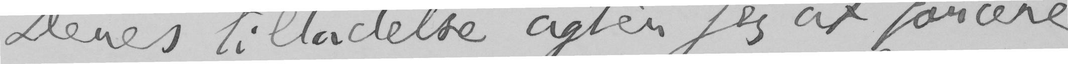Deres tilladelse agter jeg at forære
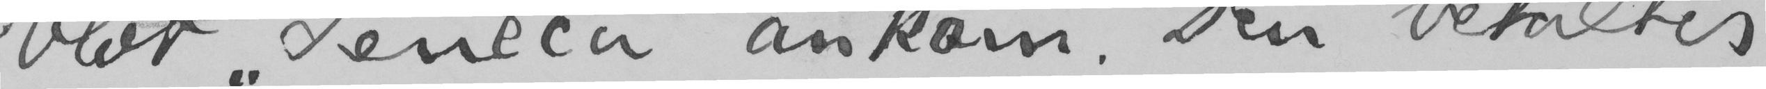blot «Seneca» ankom. Den betaltes
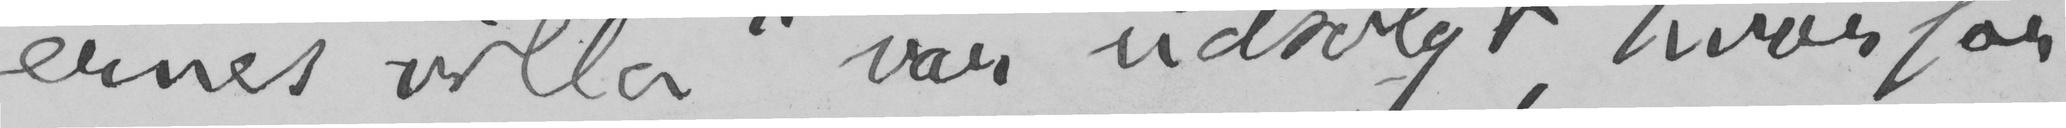ernes villa» var udsolgt, hvorfor
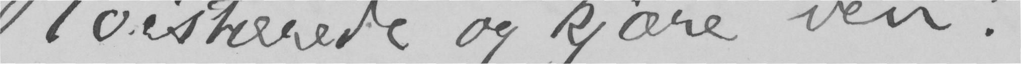Høistærede og kjære ven!
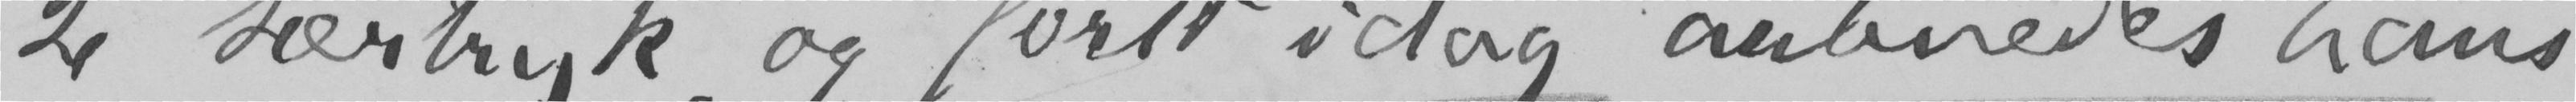2 særtryk, og først i dag aabnedes hans
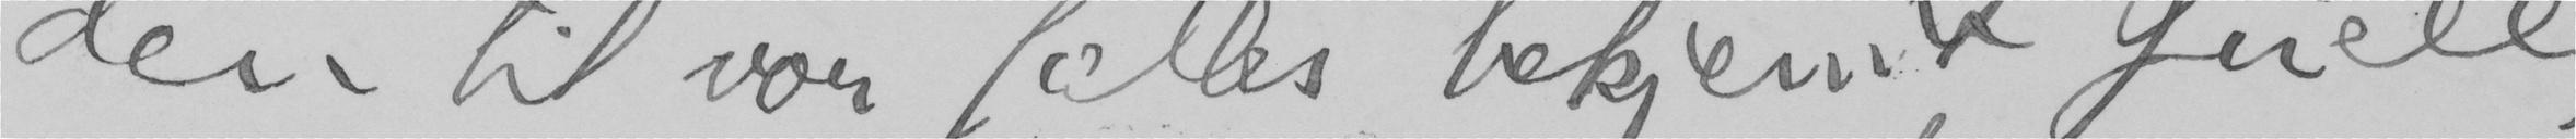den til vor fælles bekjendt Juell,
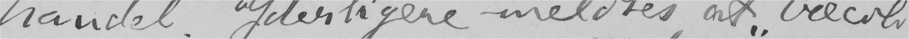handel. Yderligere meldtes at «Cæcili-
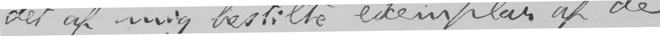det af mig bestilte exemplar af de
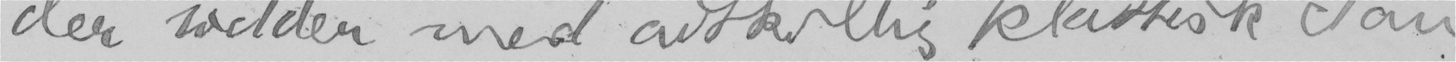der sidder med adskillig klassisk dan-
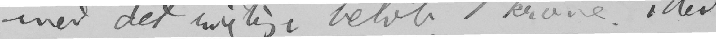med det rigtige beløb, 1 krone. Med
